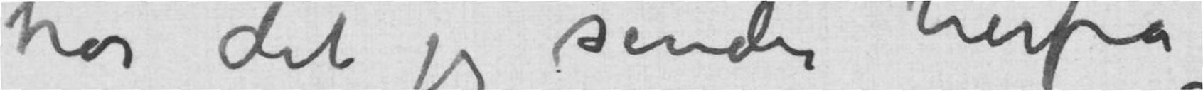tror det jeg sender herfra
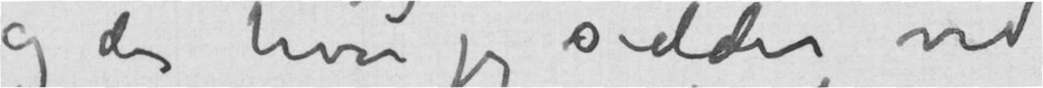og der hvor jeg sidder ved
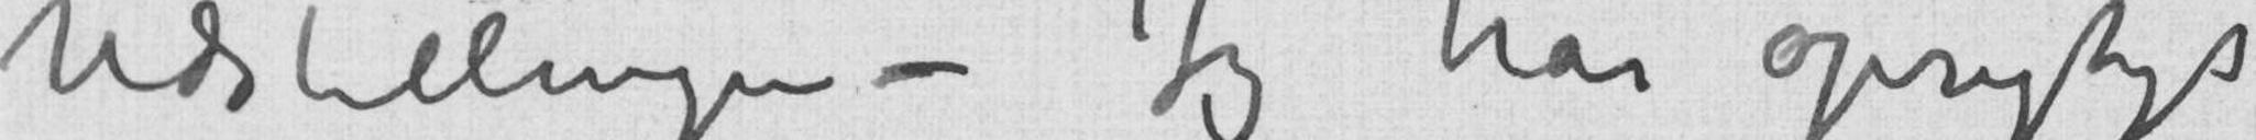Udstillingen – Jeg har oprigtig
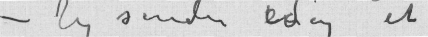– Jeg sender idag et
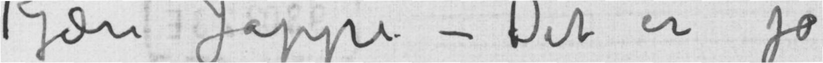Kjære Jappe! Det er jo
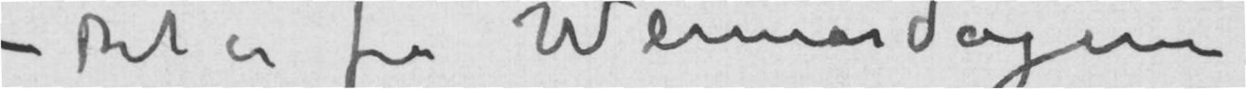– Det er fra Weimardagene
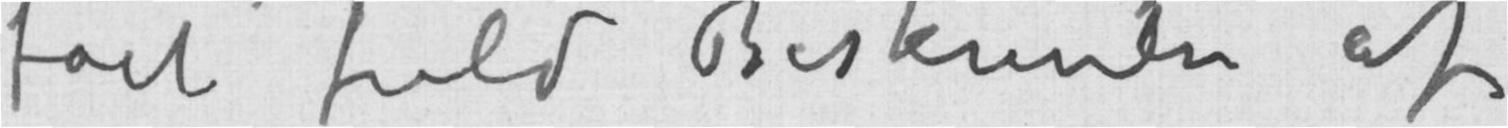fået fuld Beskrivelse af
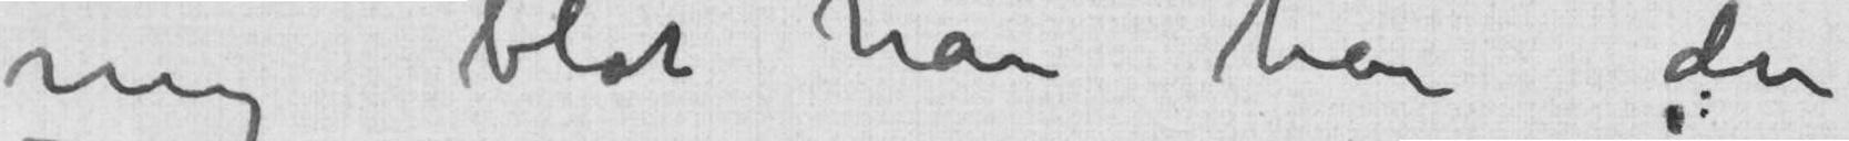mig blot han har den
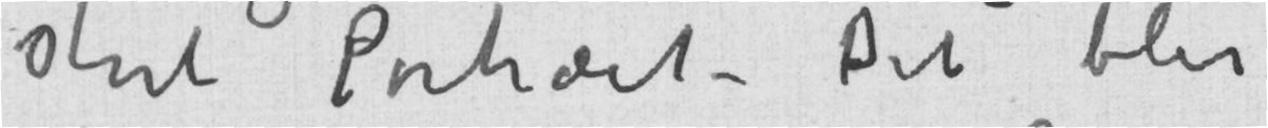stort Portræt – Det blir
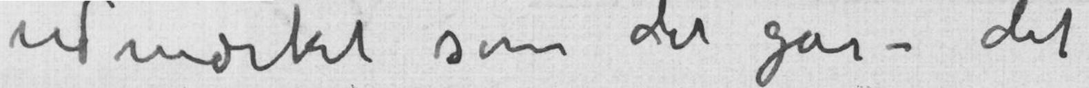udmærket som det går – det
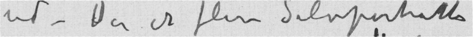ud – Der er flere Selvportrætter
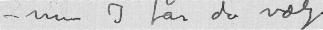– men I får da vælge
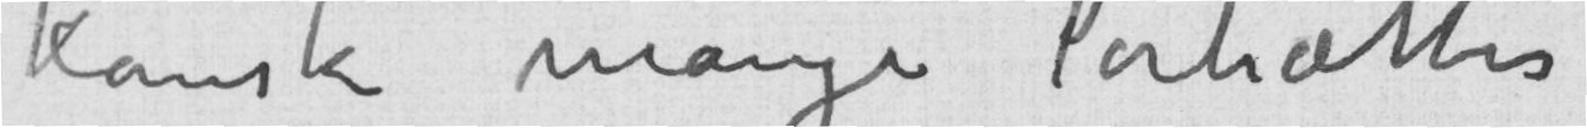kanske mange Portrætter
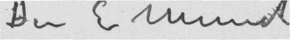Din E Munch
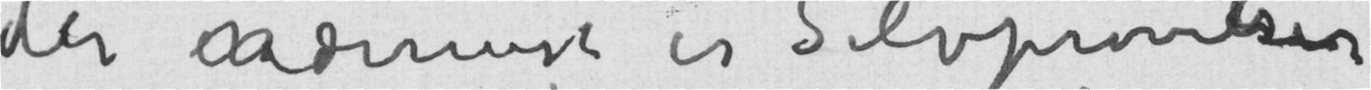der nærmest er Selvprøvelser
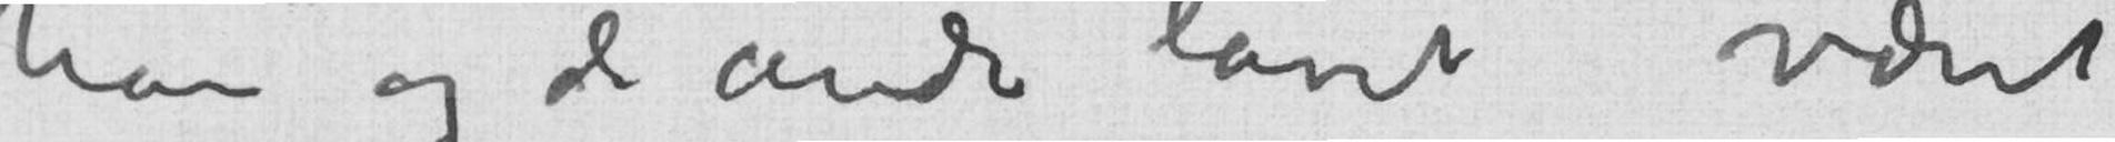han og de andre lavet været
